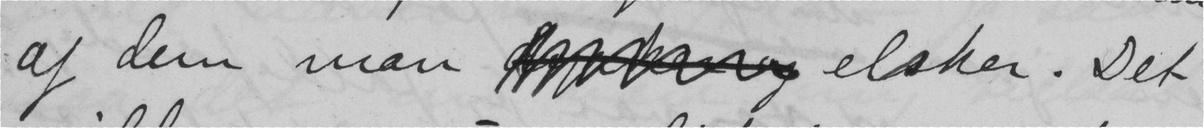af dem man  elsker. Det
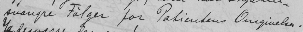svangre Følger for Patientens Omgivelser.
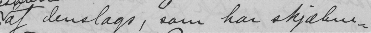af denslags, som har skjæbne-
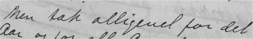Men tak alligevel for det
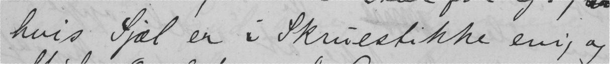hvis Sjæl er i Skruestikke evig og
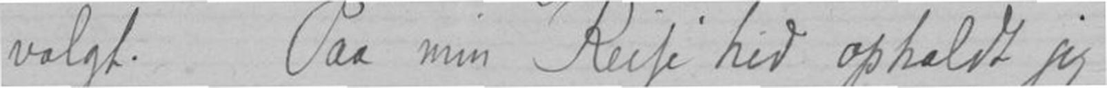valgt. Paa min Reise hid opholdt jeg
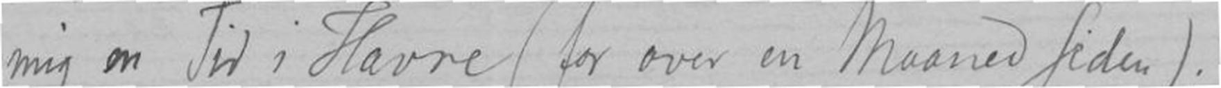mig en Tid i Havre (for over en Maaned siden);
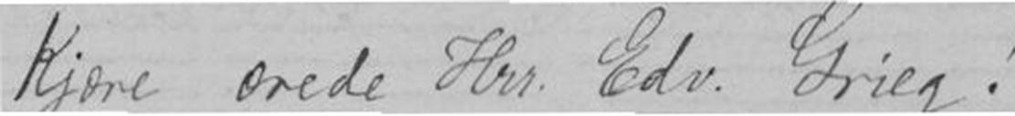Kjære ærede Hrr. Edv. Grieg!
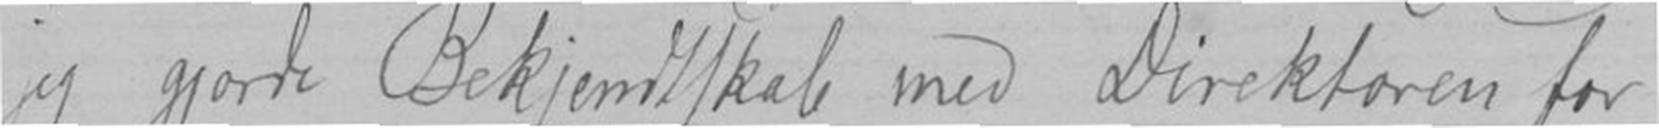jeg gjorde Bekjendtskab med Direktøren for
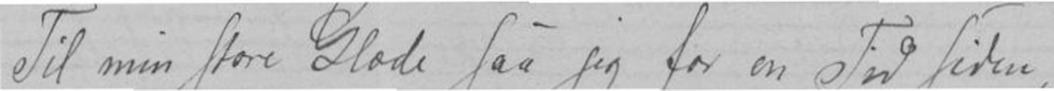Til min Glæde saa jeg for en tid siden,
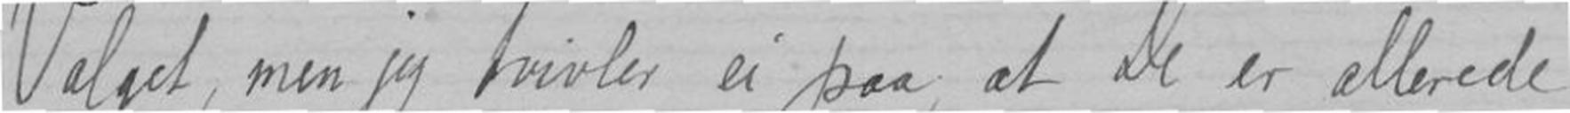Valget, men jeg tvivler ei paa, at De er allerede
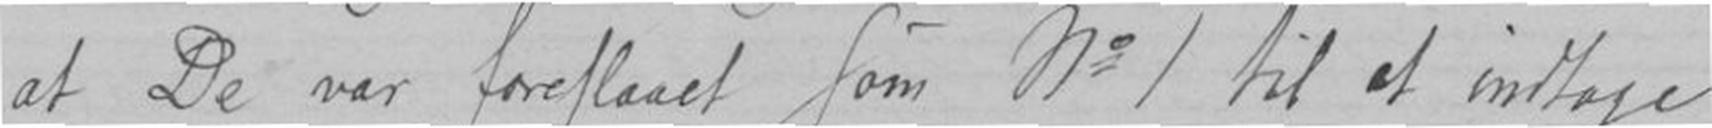at De var foreslaaet som No 1 til at indtage
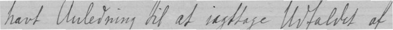havt Anledning til at iagtage Udfaldet af
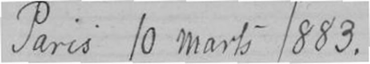Paris 10 Marts 1883.
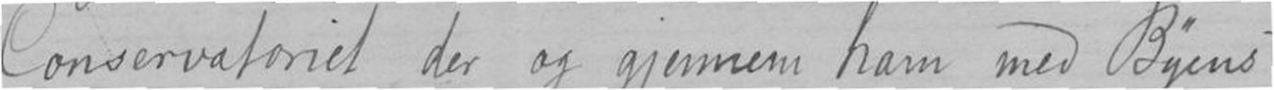Concervatoriet der og gjennem ham med Byens
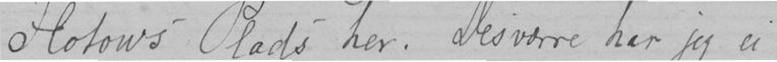Flotows Plads her. Desværre har jeg ei
